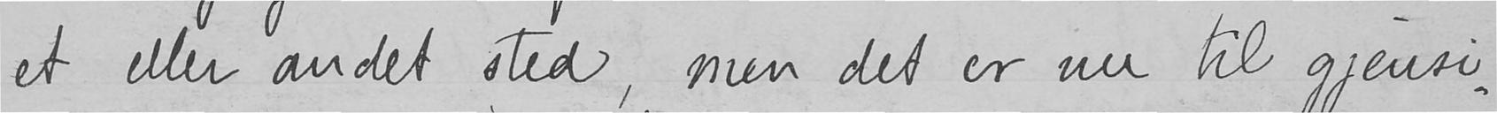et eller andet sted, men det er nu til gjensi-
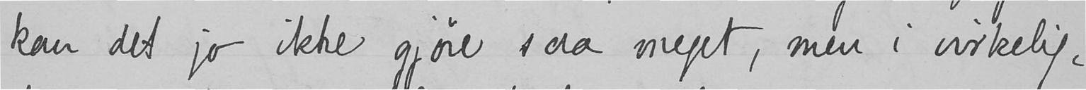kan det jo ikke gjøre saa meget, men i virkelig-
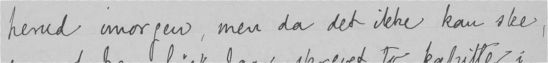herud imorgen, men da det ikke kan ske,
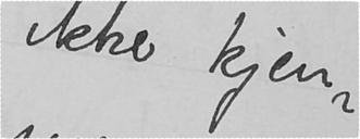ikke kjen-
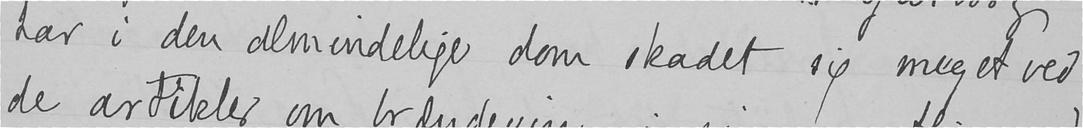har i den almindelige dom skadet sig meget ved
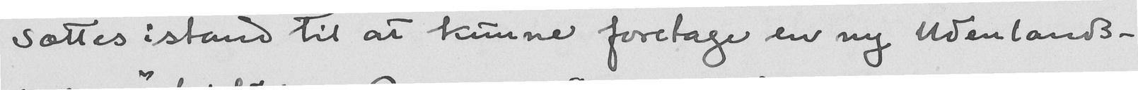sættes istand til at kunne foretage en ny Udenlands-
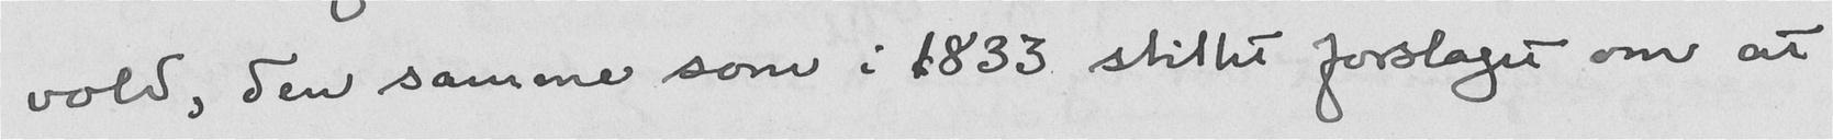vold, den samme som i 1833 stillet forslaget om at
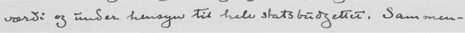værdi og under hensyn til hele statsbudgettet. Sammen-
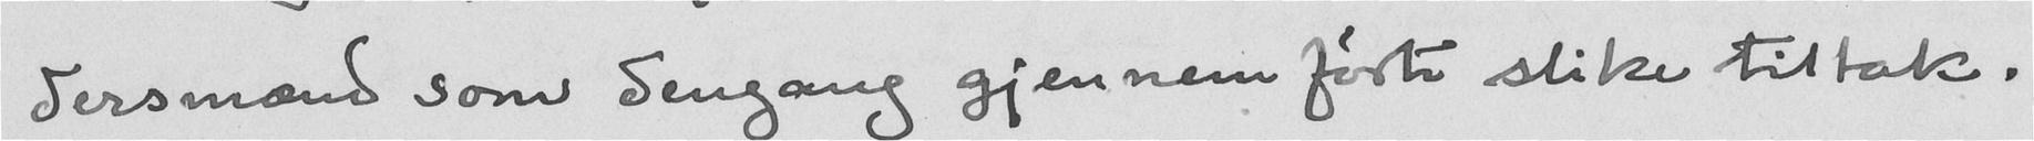dersmænd som dengang gjennemførte slike tiltak.
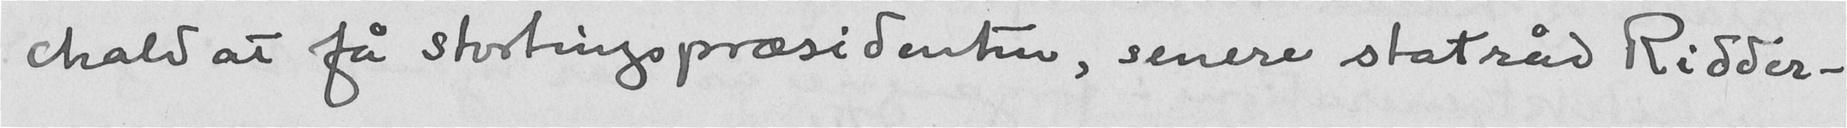chald at få stortingspræsidenten, senere statråd Ridder-
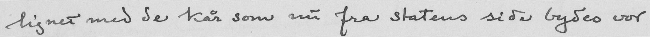lignet med de kår som nu fra statens side bydes vor
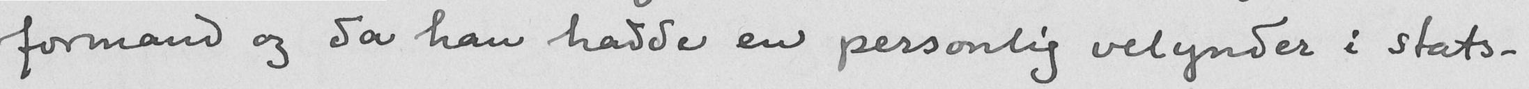formand og da han hadde en personlig velynder i stats-
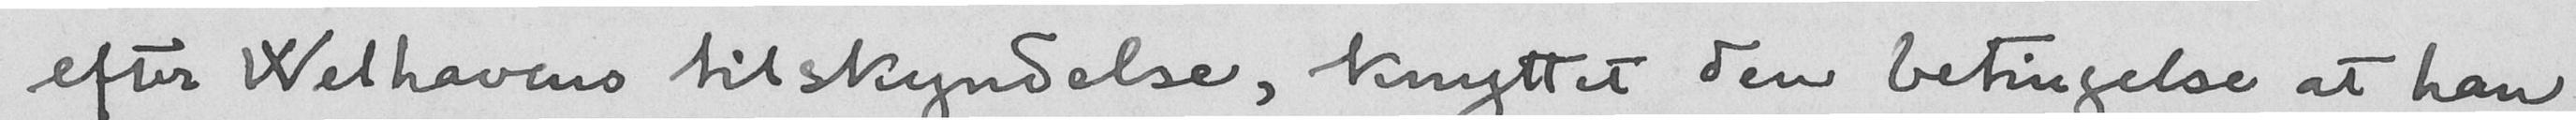efter Welhavens tilskyndelse, knyttet den betingelse at han
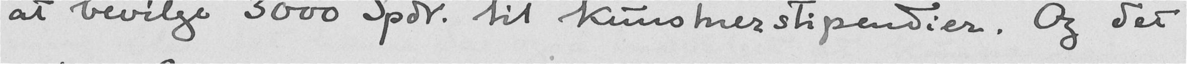at bevilge 3000 spdr. til kunstnerstipendier. Og det
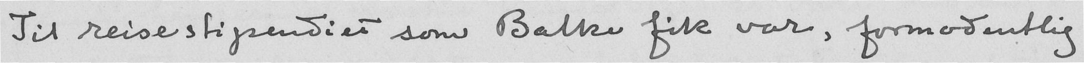Til reisestipendiet som Balke fik var, formodentlig
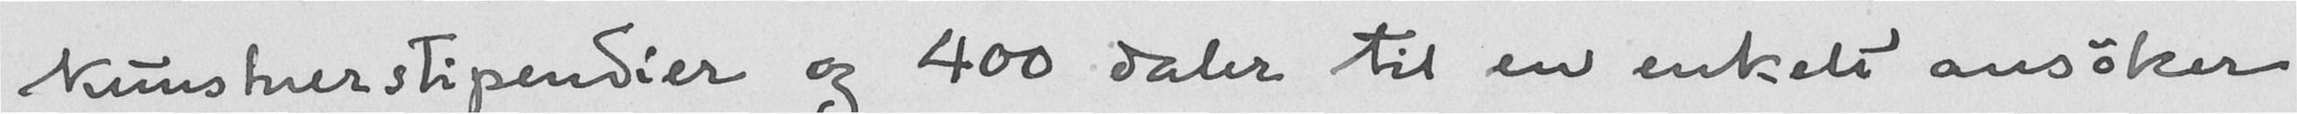kunstnerstipendier og 400 daler til en enkelt ansøker
